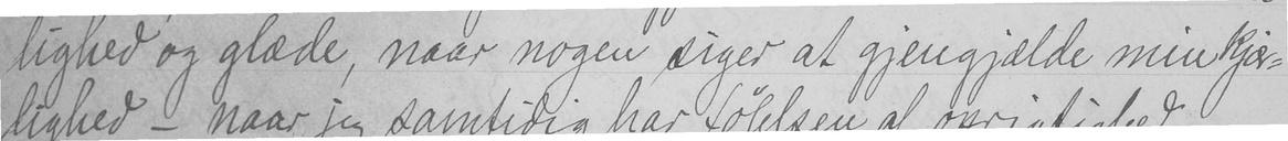lighed og glæde, naar nogen siger at gjengjælde min kjær-
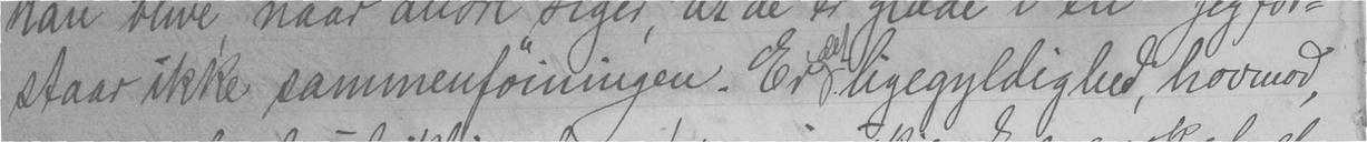staar ikke sammenføingen. Er det ligegyldighed, hovmod,
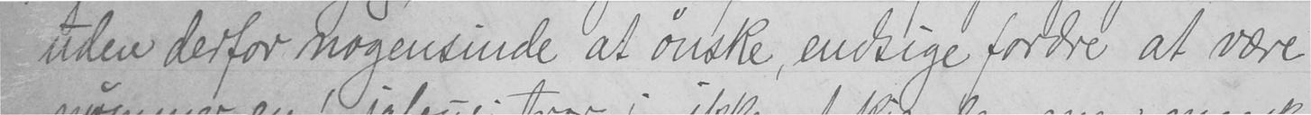uden derfor nogensinde at ønske, endsige fordre at være
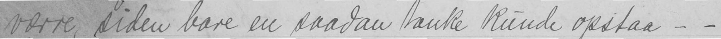værre, siden bare en saadan tanke kunde opstaa - -
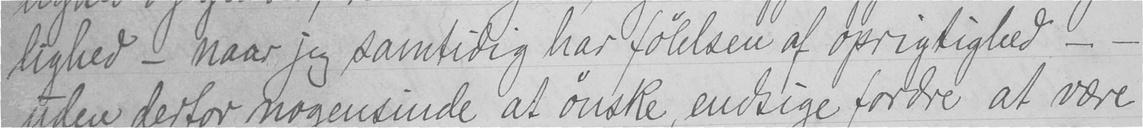lighed - naar jeg samtidig har følelsen af oprigtighed - -
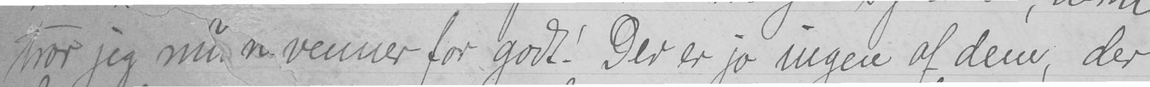tror jeg mine venner for godt! Der er jo ingen af dem, der
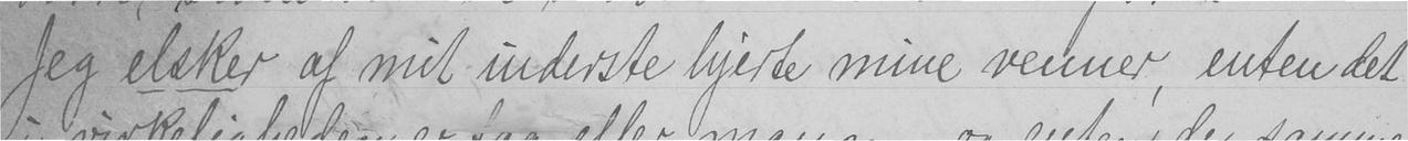Jeg elsker af mit inderste hjerte mine venner, enten det
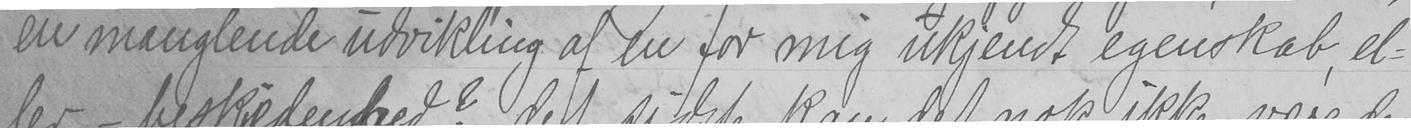en manglende udvikling af en for mig ukjendt egenskab, el-
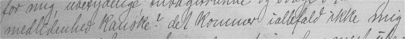medlidenhed kanske? det kommer iallefald ikke mig
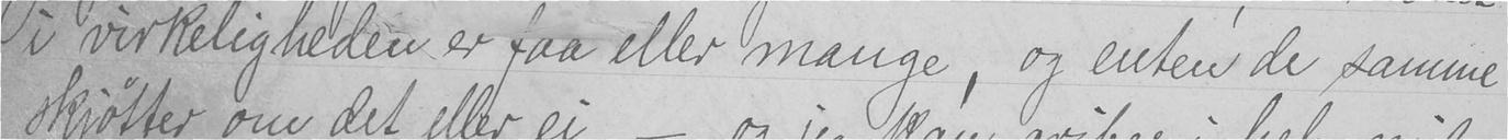i virkeligheden er faa eller mange, og enten de samme
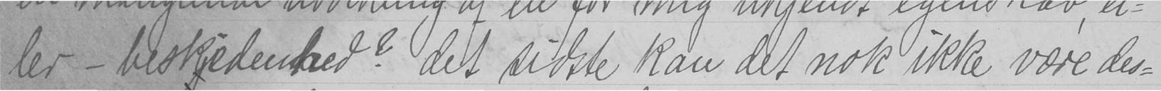ler beskjedenhed? det sidste kan det nok ikke være des-
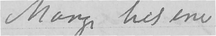Mange hilsener
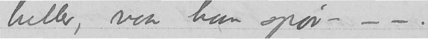heller, naar han spør –- –- –-
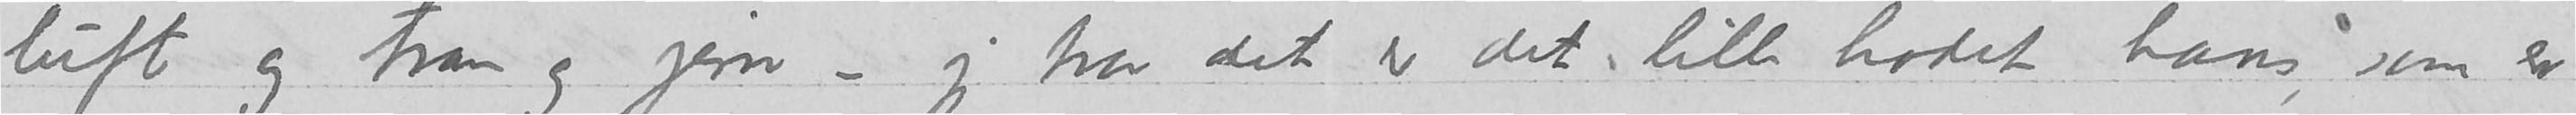luft og tran og jern – jeg tror det er det lille hodet hans, som er
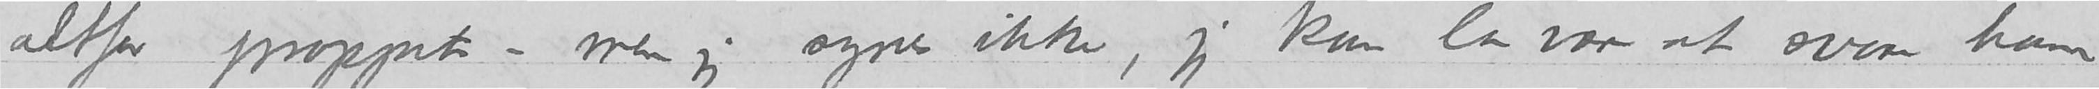altfor proppet – men jeg synes ikke, jeg kan la være at svare ham
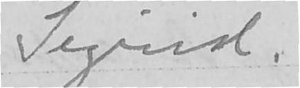Ingrid
